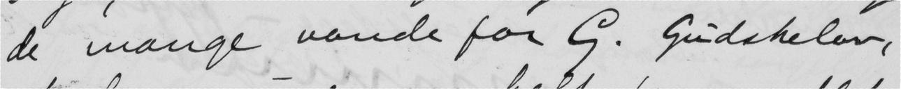de mange vonde for G. Gudskelov,
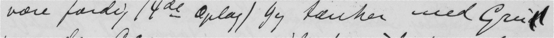være færdig (4de Oplag) Jeg tænker med Gru
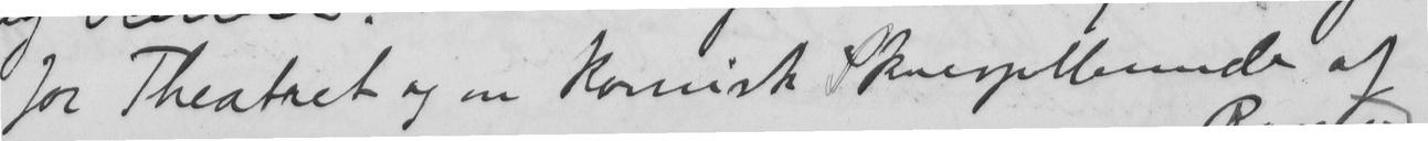for Theatret og en komisk Skuespillerinde af
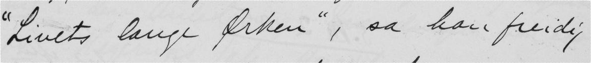"Livets lange Ørken", sa han freidig
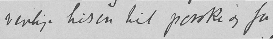venlige hilsen til paaske og for
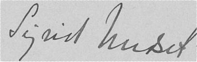Sigrid Undset.
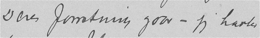Deres forretning gaar - jeg haaber
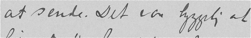at sende. Det var hyggelig at
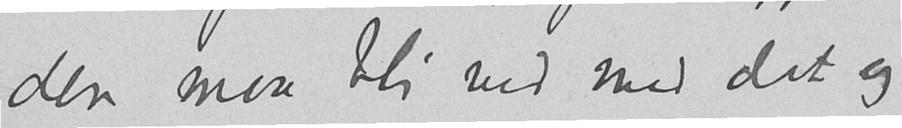den maa bli ved med det og
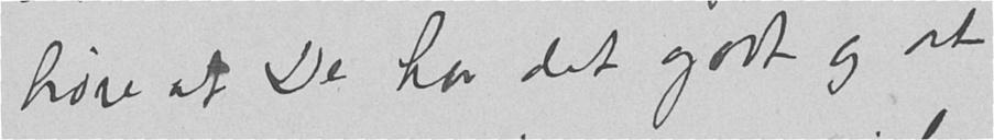høre at De har det godt og at
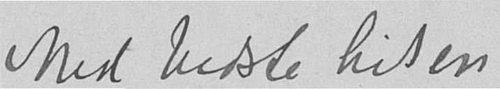Med bedste hilsen
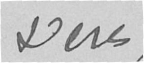Deres
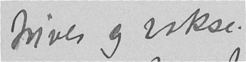trives og vokse.
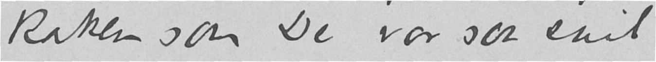kaken som De var saa snil
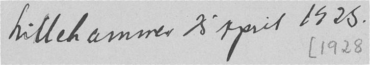Lillehammer 25 april 1925.
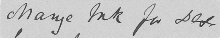Mange tak for Deres
